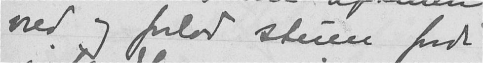vred og forlod stuen fordi
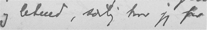og letned, særlig tror jeg for
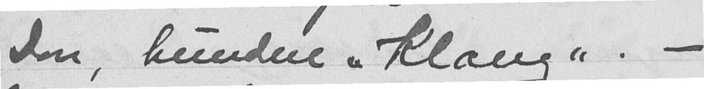Don, hundere "Klang". -
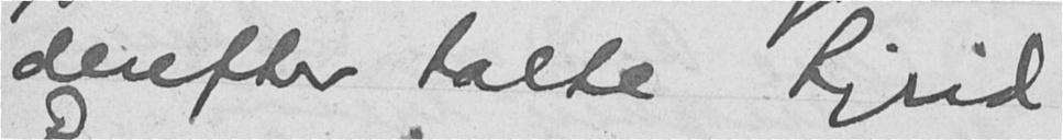derefter talte Sigrid,
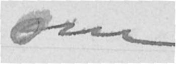om
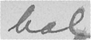bal
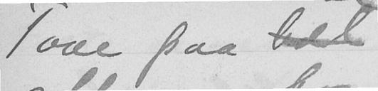Tove paa bdl
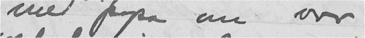med papa om vor
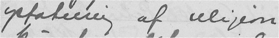opfatning af religion
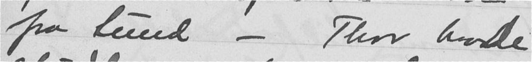fra Sund - Thor havde
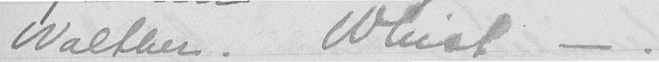Walther. Whist - .
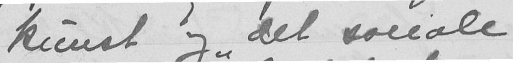kunst og "det sociale
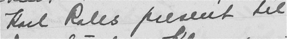Karl Rolls present til
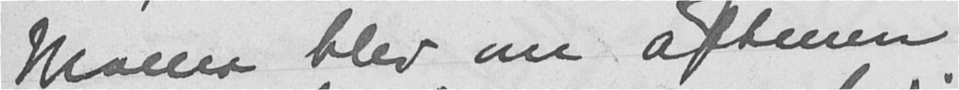Mama blev om aftenen
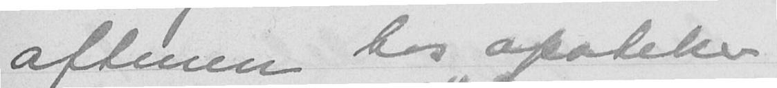aftenen hos apoteker
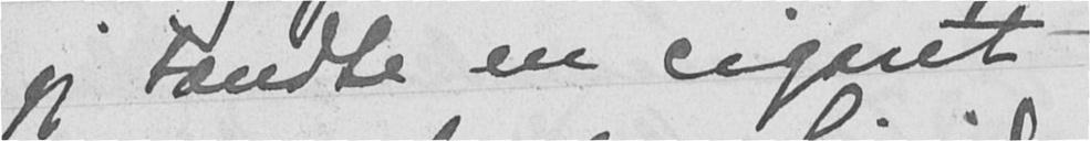jeg tændte en cigaret
